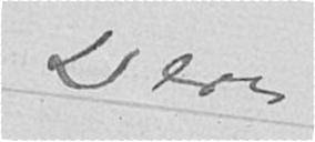Deres
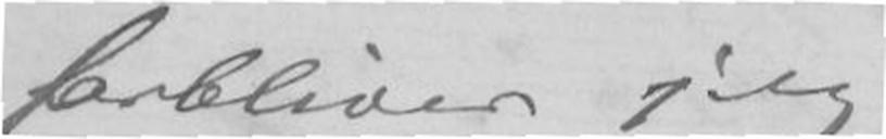forbliver jeg
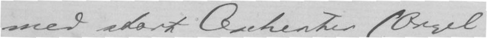med stort Orchester (orgel
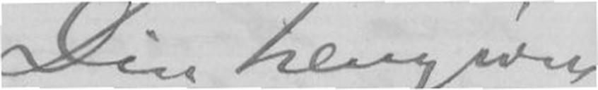Din hengivne
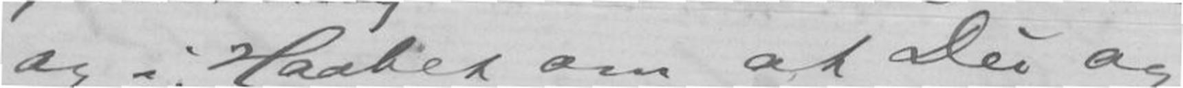og i Haabet om at Du og
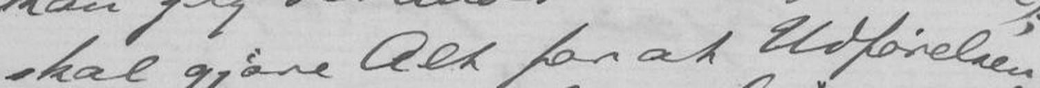skal gjøre Alt for at Udførelsen
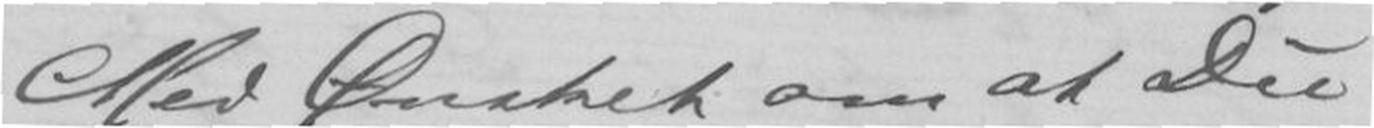Med Ønsket om at Du
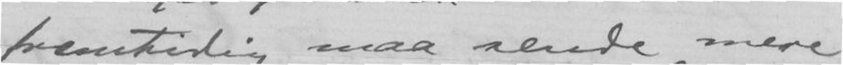fremtidig maa sende mere
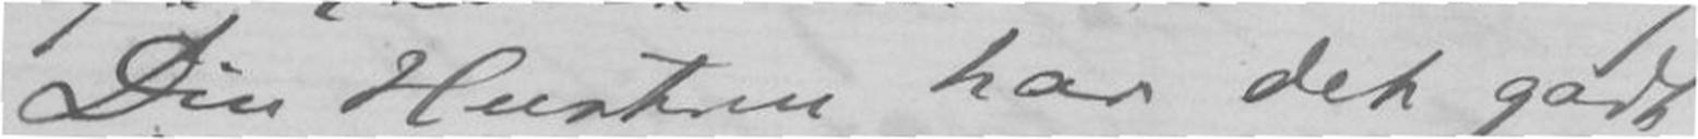Din hustru har det godt
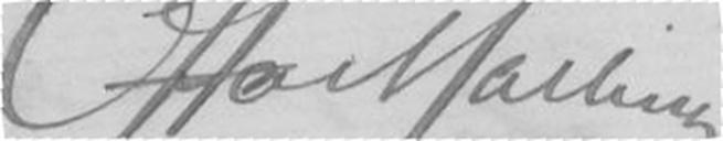Otto Malling
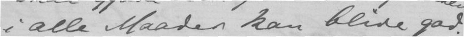i alle Maader kan blive god.
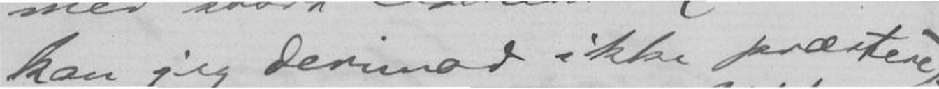kan jeg derimod ikke præstere);
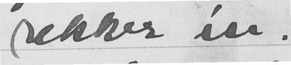rekker in.
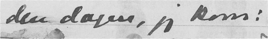den dagen, jeg kom:
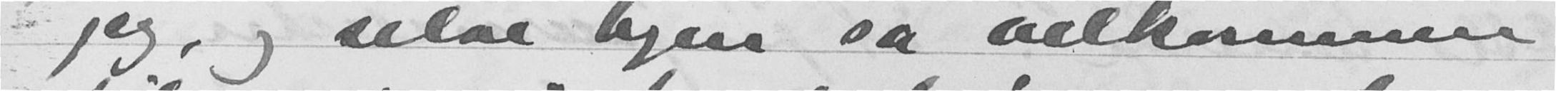jeg, og selve byen sa velkommen
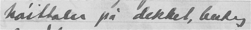høittaler pa dekket, besteg
